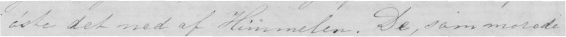øste det ned af Himmelen. De, som morede
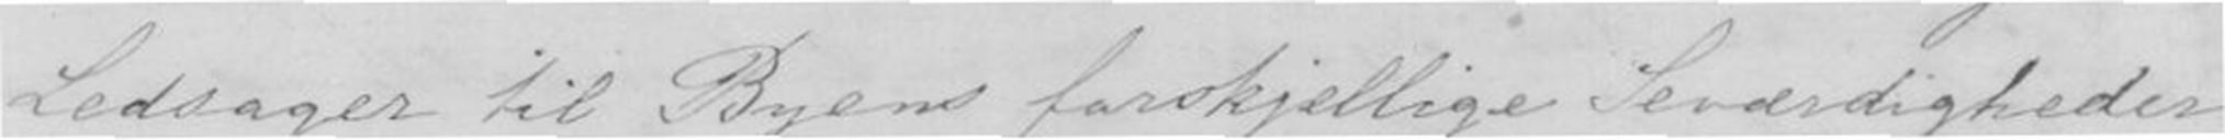Ledsager til Byens forskjellige Seværdigheder
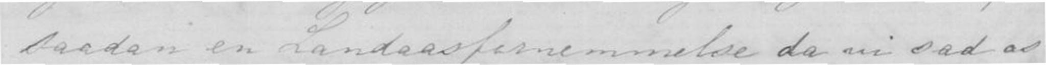saadan en Landaasfornemmelse da vi sad os
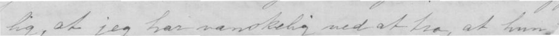lig, at jeg ikke har vanskelig ves at tro, at hun
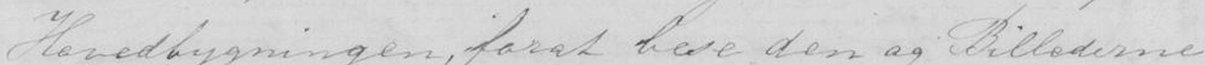Hovedbygningen, forat bese den og Billederne
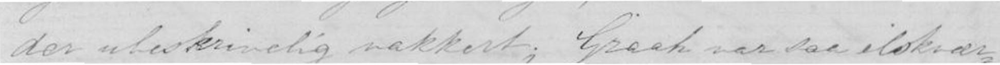der ubeskrivelig vakkert; Graah var saa elskvær-
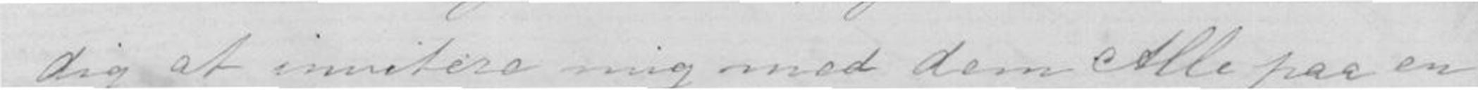dig at invitere mig med dem Alle paa en
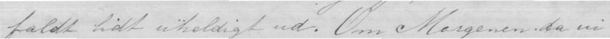faldt lidt uheldigt ud. Om Morgenen da vi
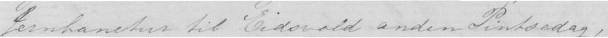Jernbanetur til Eidsvold anden Pintsedag,
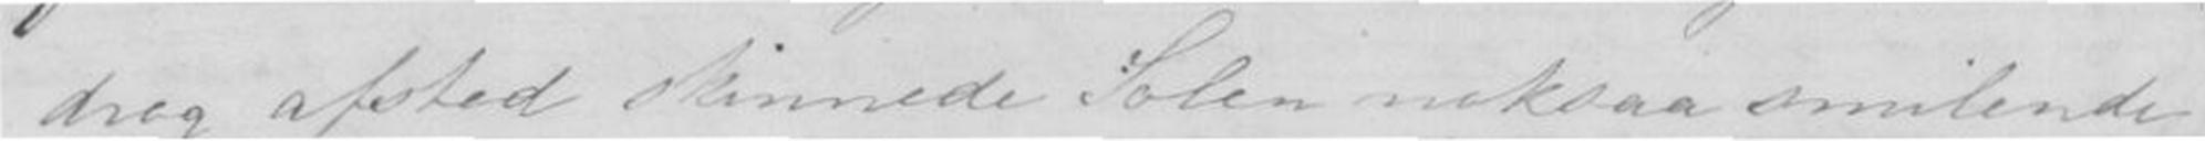drog afsted skinnede Solen noksaa smilende
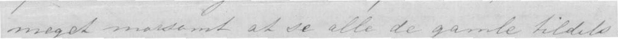meget morsomt at se alle de gamle tildels
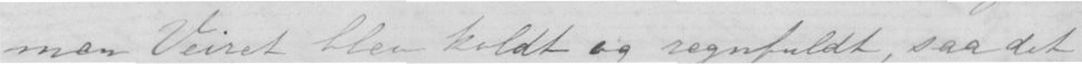men Veiret blev koldt og regnfuldt, saa det
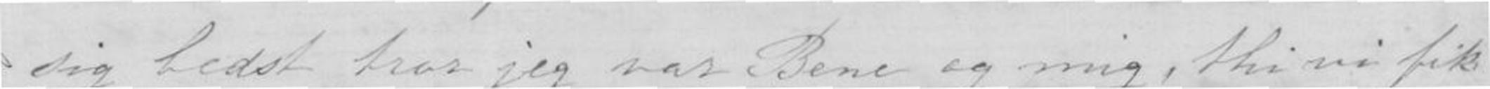sig bedst tror jeg var Bene og mig, thi vi fik
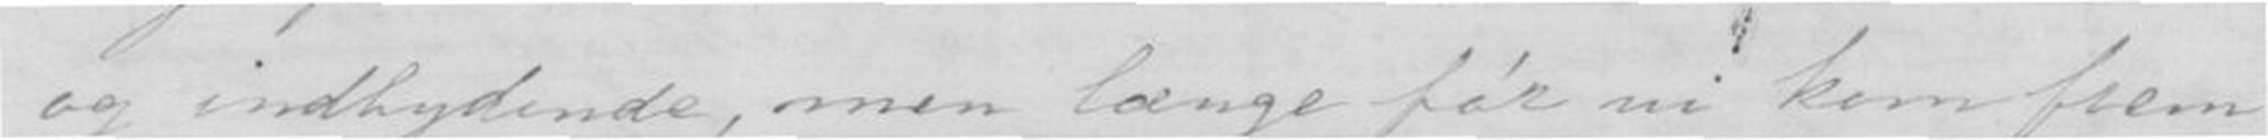og indbydende, men længe før vi kom frem
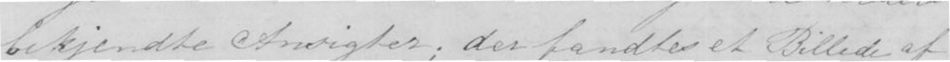bekjendte Ansigter, der fandtes et Billede af
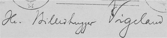Hr. Billedhugger Vigeland
